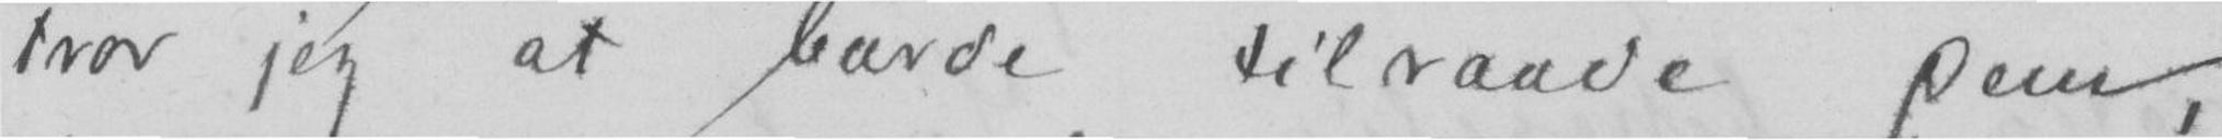tror jeg at burde tilraade Dem,
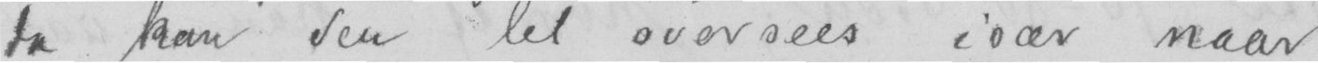da kan den let oversees især naar
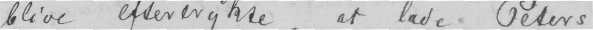blive eftertrykte, at lade Peters
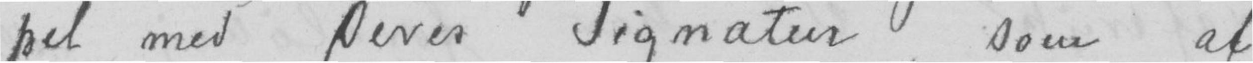pel med Deres Signatur, som af
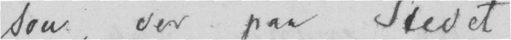son, der paa Stedet
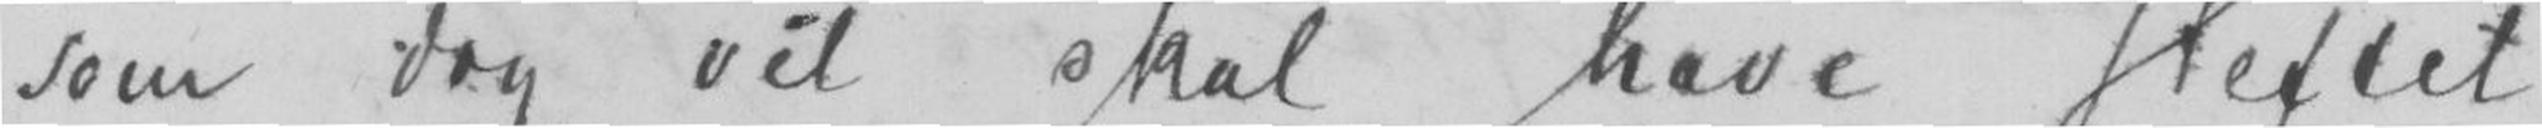som dag vil skal have Heftet
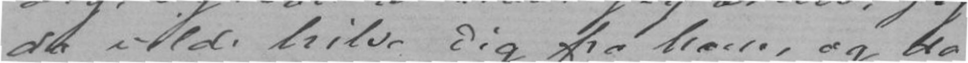da vilde hilse Dig fra ham, og da
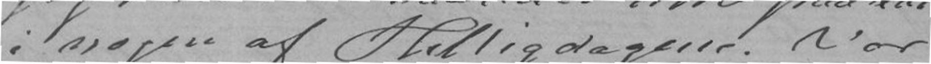i nogen af Helligdagene. Vor
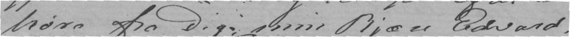høre fra Dig min kjære Edvard,
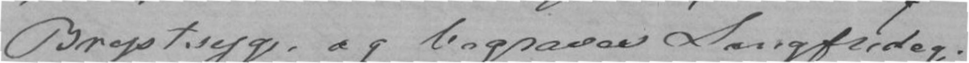Brystsyge, og begraves Langfredag.
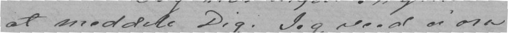at meddele Dig. Jeg veed ei om
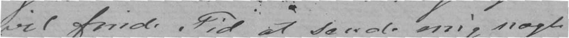vil finde Tid at sende mig nogle
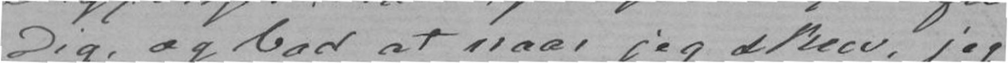Dig, og bad at naar jeg skrev, jeg
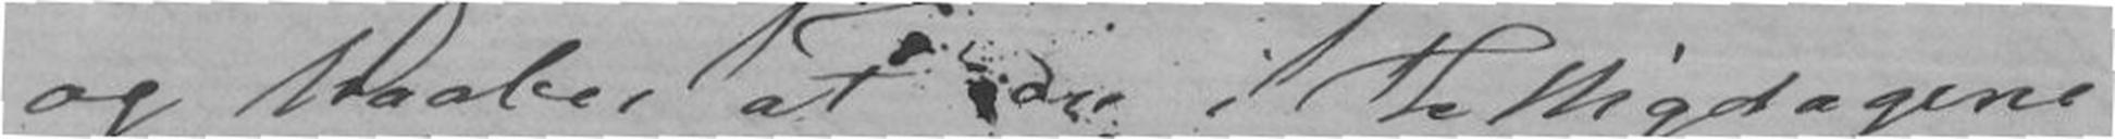og haaber at Du i Helligdagene
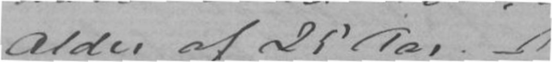Alder af 25 Aar.
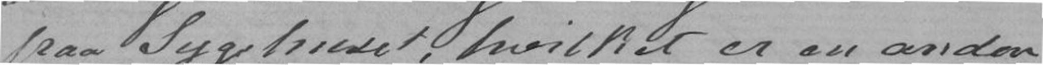paa Sygehuset, hvilket er en anden
